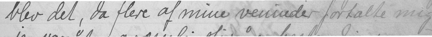blev det, da flere af mine veninder fortalte mig
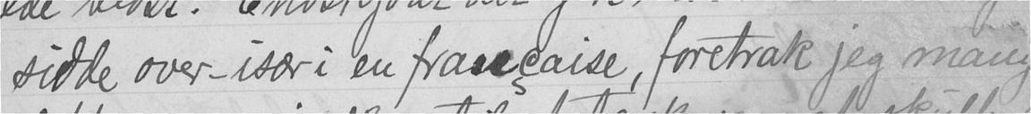sidde over-især i en française, foretrak jeg mang
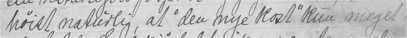høist naturlig, at "den nye kost" kun meget
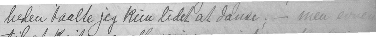heden taalte jeg kun lidet at dause; - men evnen
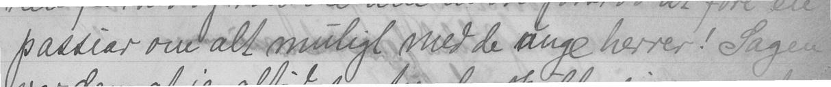passiar om alt muligt med de unge herrer! Sagen
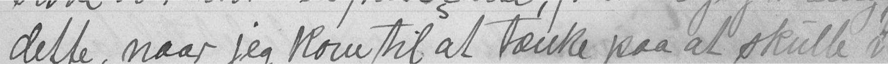dette, naar jeg kom til at tænke paa at skulle i
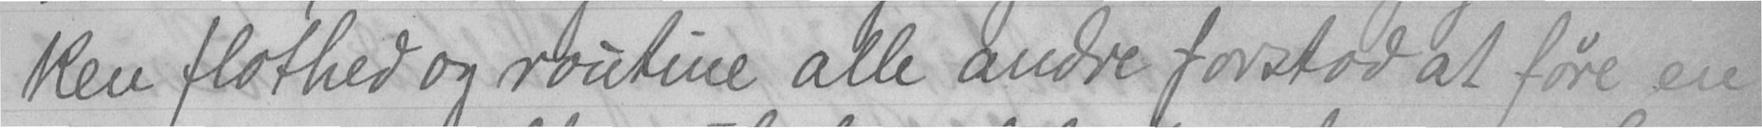ken flothed og routine alle andre forstod at føre en
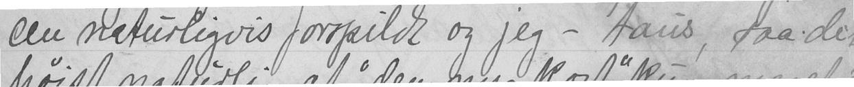cen naturligvis forspildt og jeg - taus, saa det som
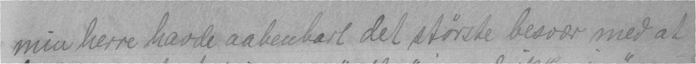min herre havde aabenbart det største besvær med at
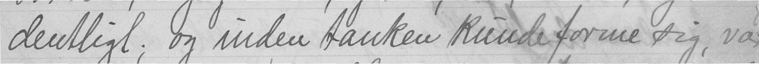dentligt; og inden tanken kunde forme sig, va
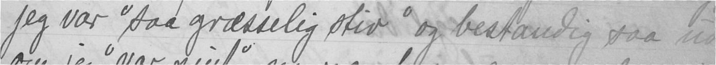jeg var "saa græsselig stiv" og bestandig saa ud
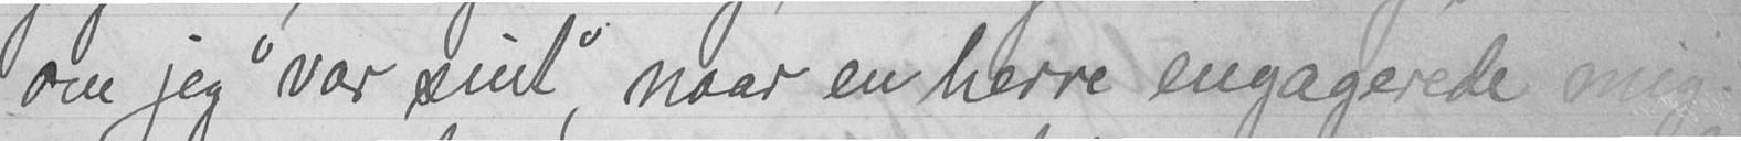om jeg " var sint" naar en herre engagerede mig.
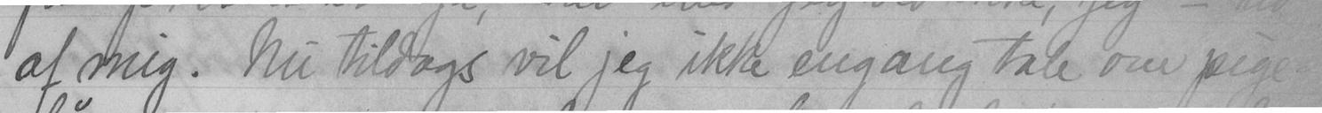af mig. Nu tildags vil jeg ikke engang tale om pige-
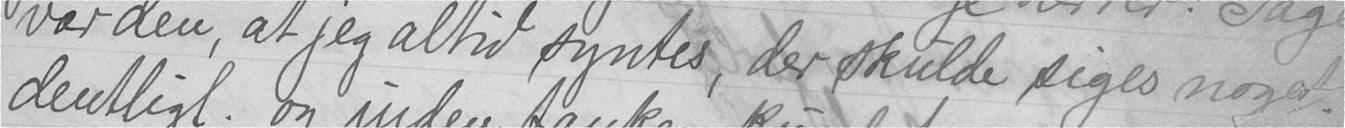var den, at jeg altid syntes, der skulde siges noget
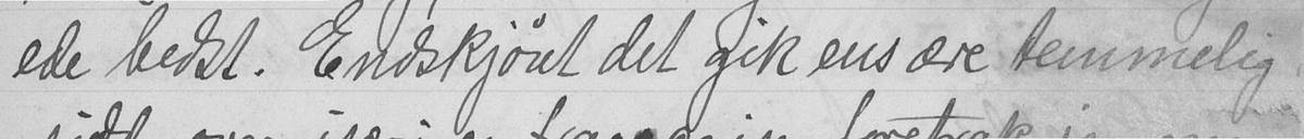ede bedst. Endskjønt det gik ens ære temmelig
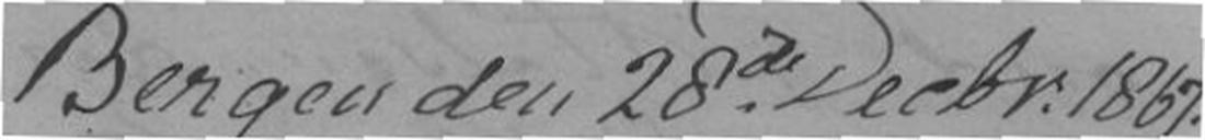Bergen den 28de Decbr. 1867.
